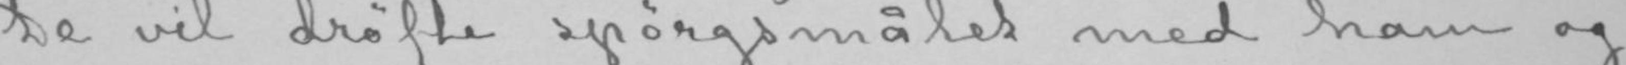De vil drøfte spørgsmålet med ham og
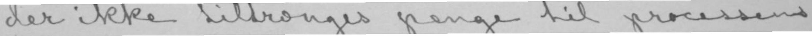der ikke tiltrænges penge til processens
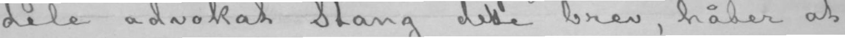dele advokat Stang dette brev, håber at
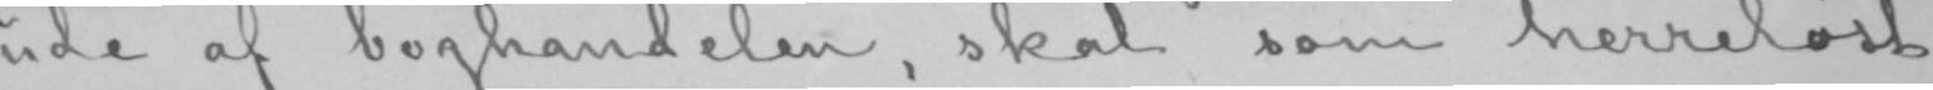ude af boghandelen, skal som herreløst
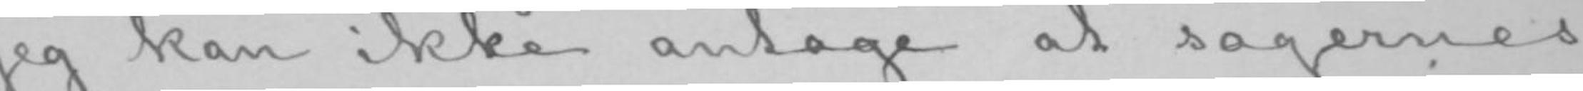Jeg kan ikke antage at sagernes
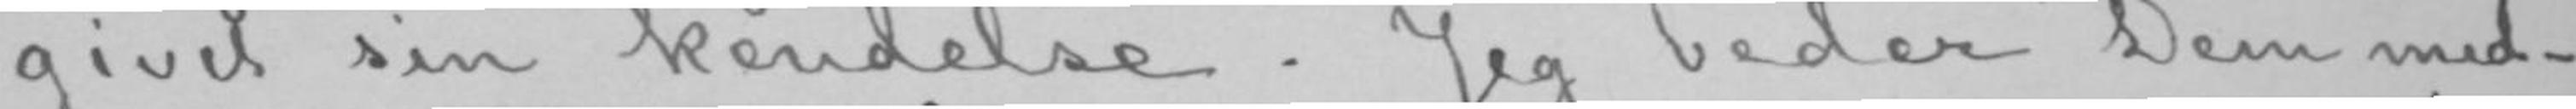givet sin kendelse. Jeg beder Dem med-
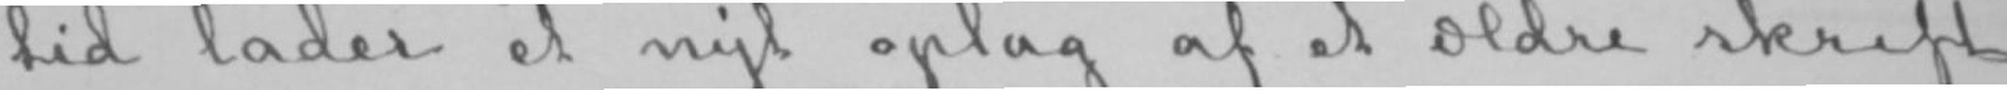tid lader et nyt oplag af et ældre skrift
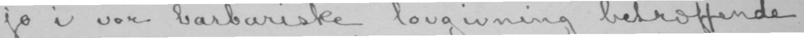jo i vor barbariske lovgivning betræffende
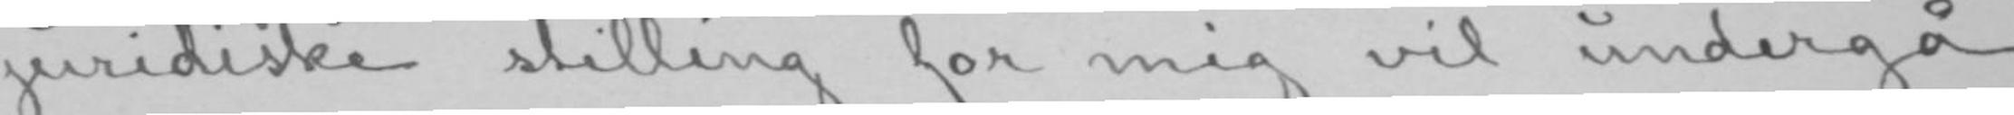juridiske stilling for mig vil undergå
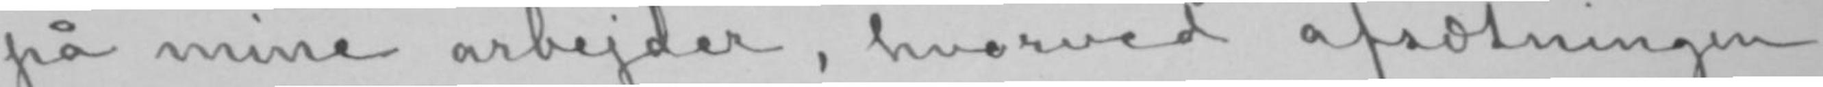på mine arbejder, hvorved afsætningen
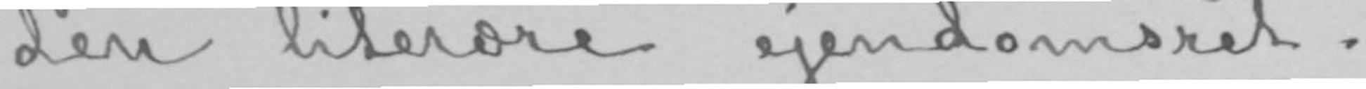den literære ejendomsret.
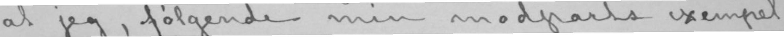at jeg, følgende min modparts exempel,
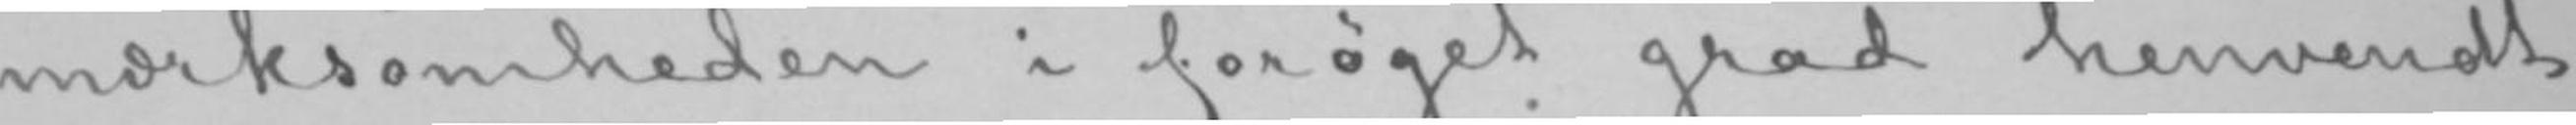mærksomheden i forøget grad henvendt
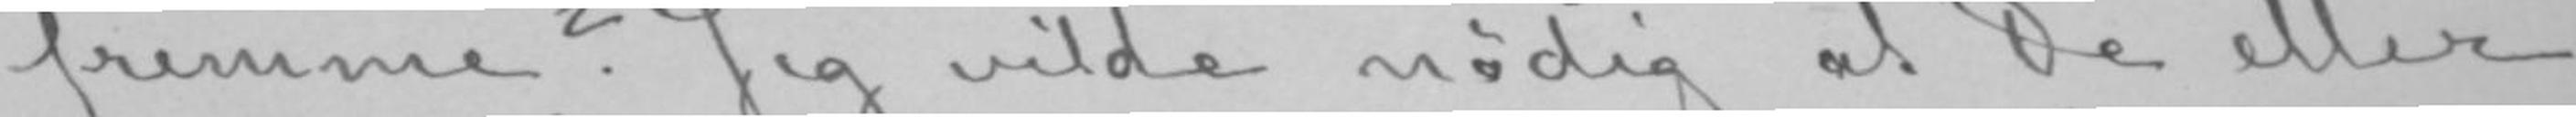fremme? Jeg vilde nødig at De eller
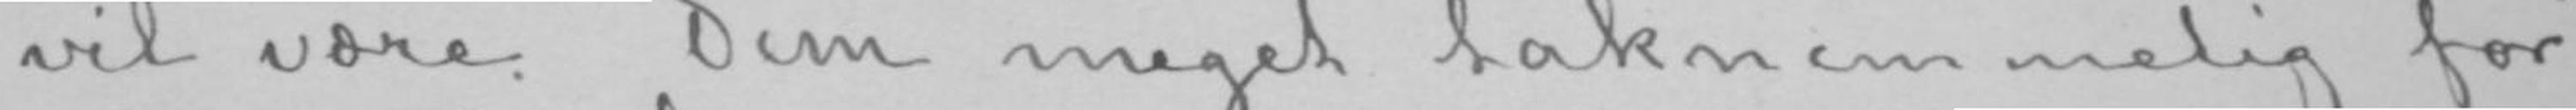vil være Dem meget taknemmelig for
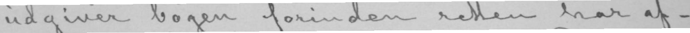udgiver bogen forinden retten har af-
